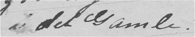i det Gamle!
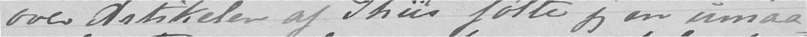over Artikelen af Thiis følte jeg en umaa
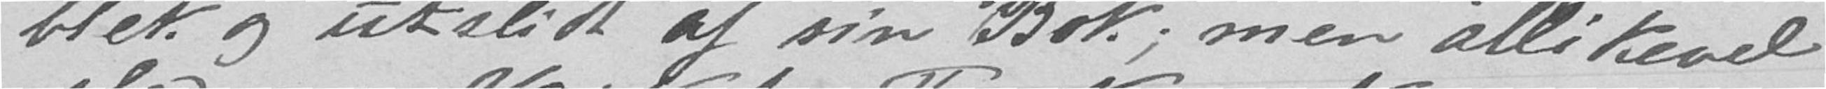blek og utslidt af sin Bok, men allikevel
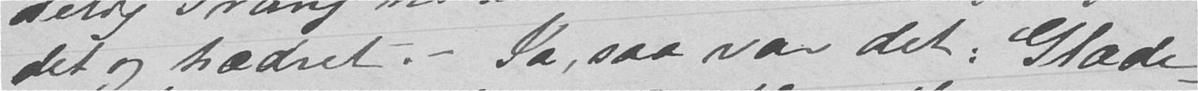det og hædret. - Ja, saa var det. Glæde-
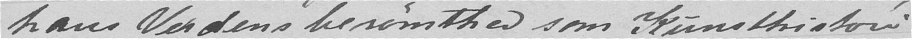hans Verdens berømthed som Kunsthistori-
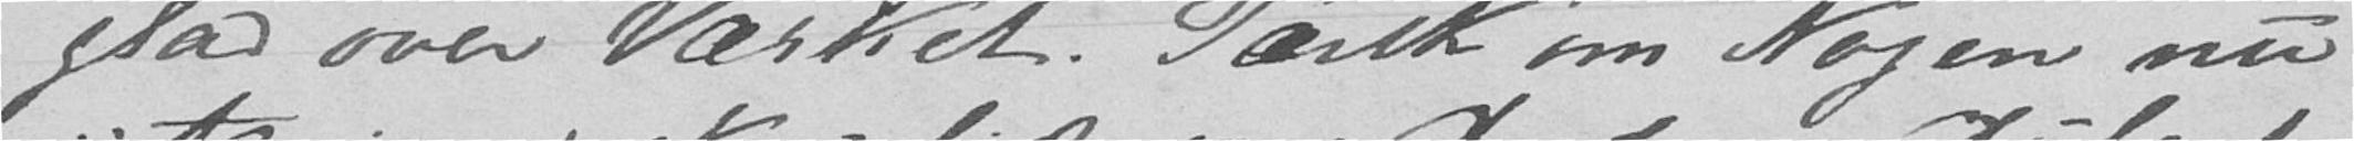glad over Værket. Tærkk om Nogen nu
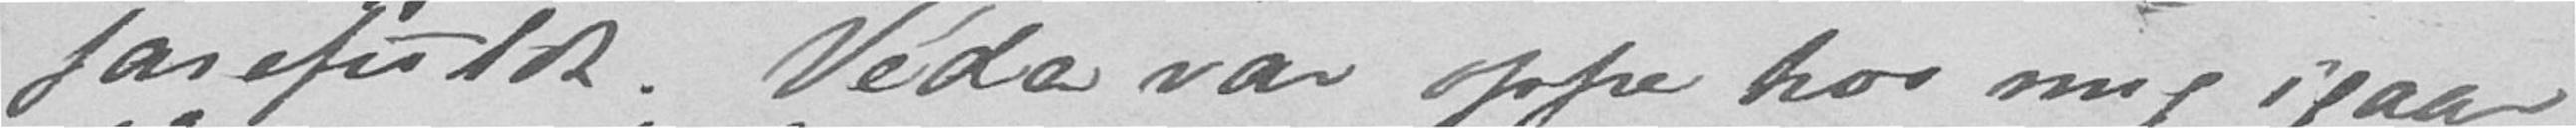farefuldt. Veda var oppe hos mig igaar
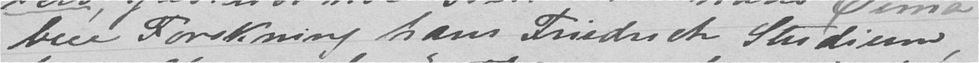bue Forskning hans Friedrich Studium,
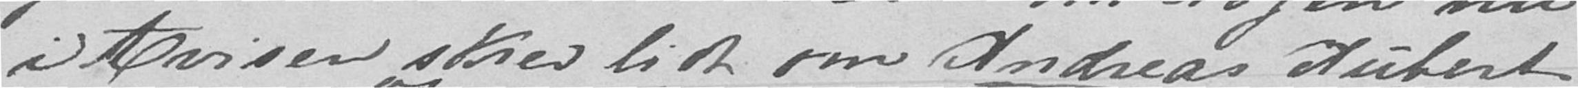i Avisen skrev lidt om Andreas Aubert
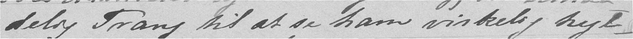delig Trang til at se ham virkelig hyl-
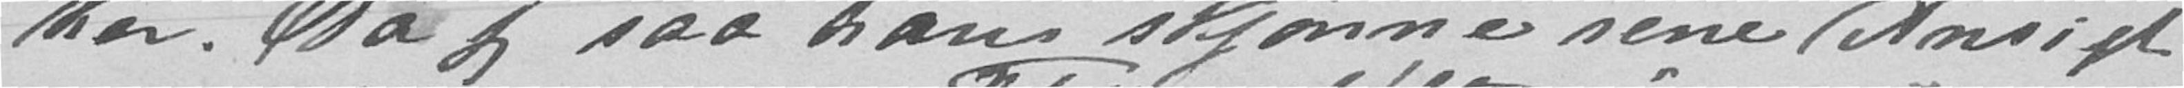ker. Da jeg saa hans skjønne rene Ansigt
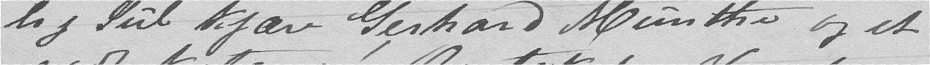lig Jul Kjære Gerhard Munthe og et
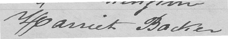Harriet Backer
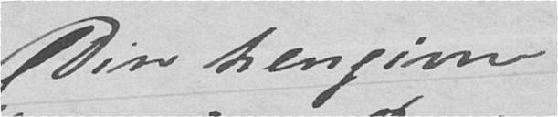Din hengivne
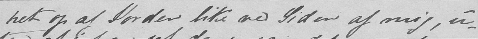ret op af Jorden like ved Siden af mig, u-
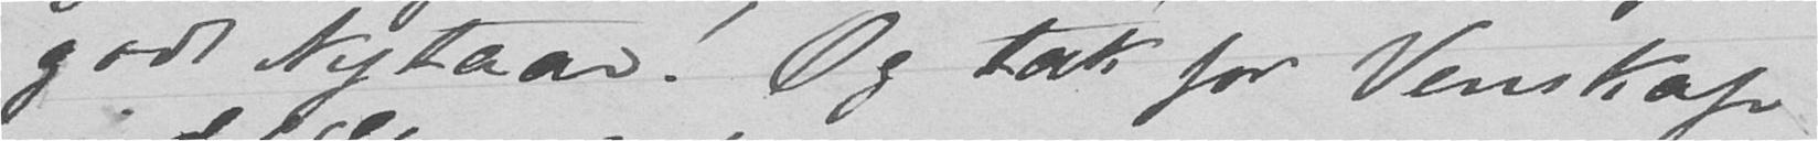godt Nytaar! Og tak for Venskap
